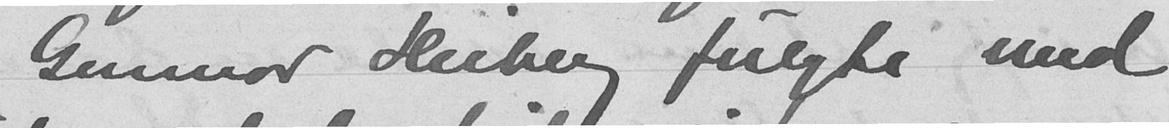Gunnar Heiberg fulgte med
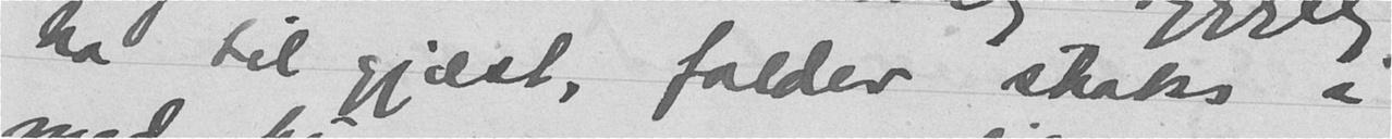ha til gjæst, falder straks i
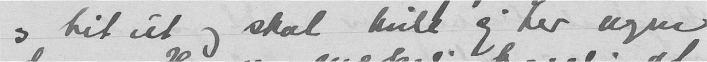os hit ut og skal hvile sig her nogen
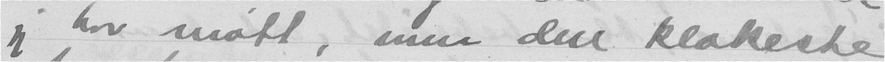jeg har møtt, men den klokeste
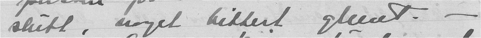slutt, noget bittert glemt. -
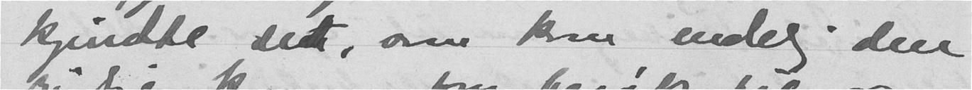kjørte det, saa kom endelig den
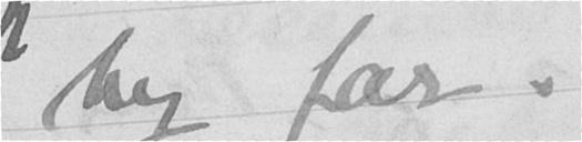by far.
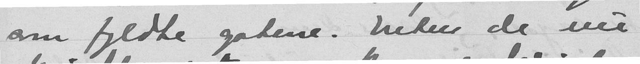som fyldte gatene. Entur de nu
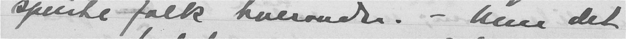spurte folk hverandre. - Men det
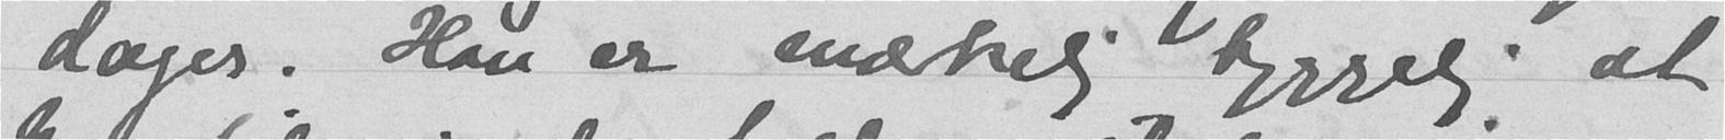dager. Han er mærkelig hyggelig at
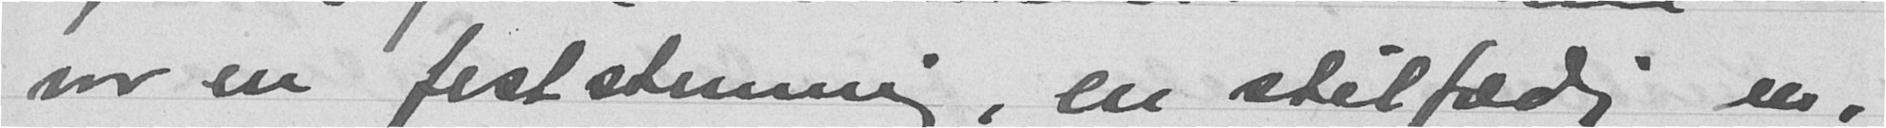var en feststemning, en stilfærdig en,
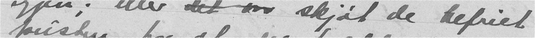igjen; eller det var skjønt de befriet
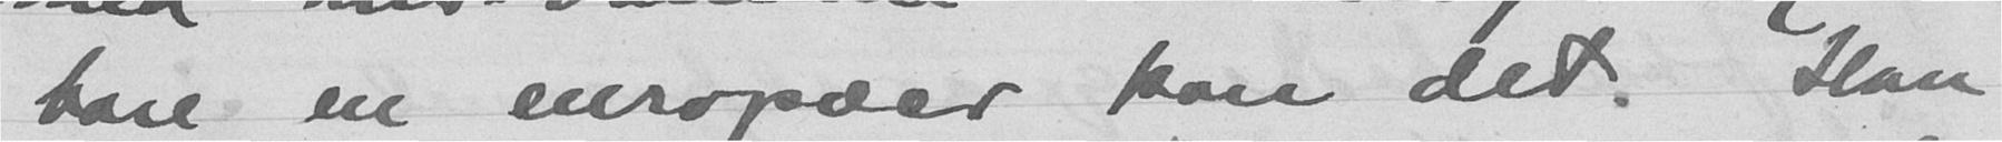bare en europæer kan det. Han
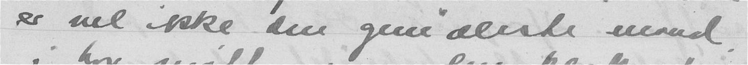er vel ikke den genialeste mand
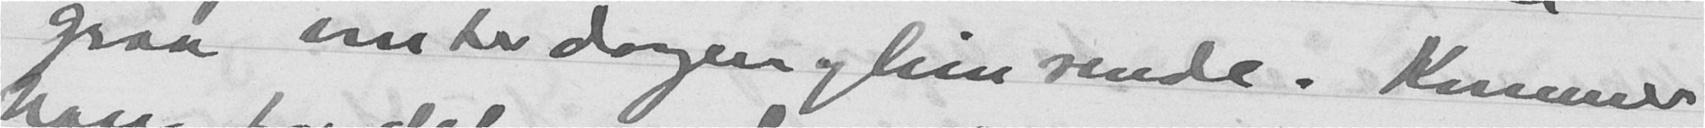graa vinterdagen glimrende. Kommer
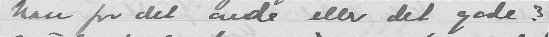han for det onde eller det gode?
